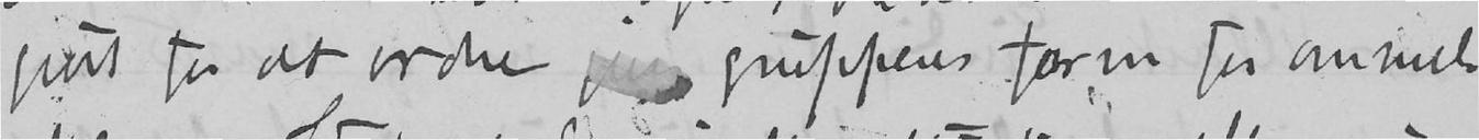gjort for at ordne jurytrolig utvisket gruppens form for anmel-
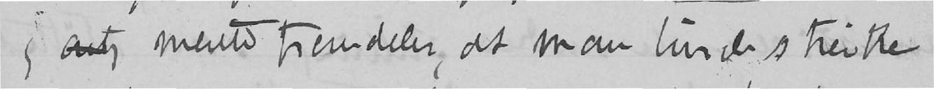og anty mente fremdeles, at man burde streike
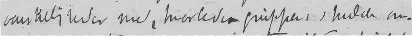vanskeligheder med, hvorledes gruppen skulde an-
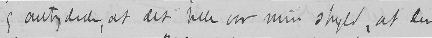og antydede, at det hele var min skyld, at der
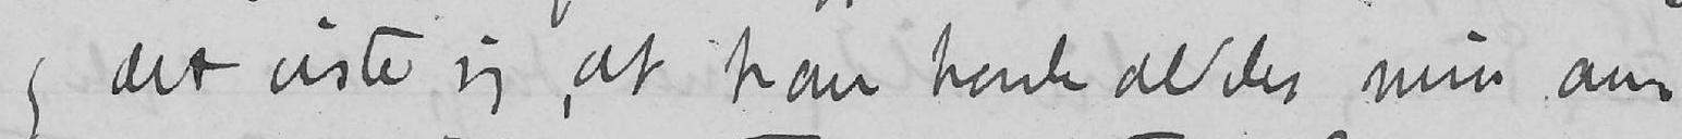og det viste sig, at han havde aldeles min ans-
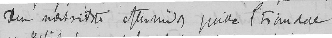Den næstsidste eftermidag gjorde Strømdal
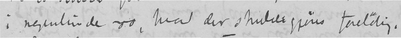i nogenlunde ro, hvad der skulde gjøres foreløbig,
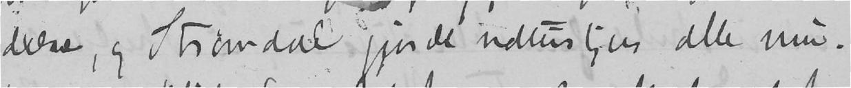delse, og Strømdal gjorde naturligvis alle mu-
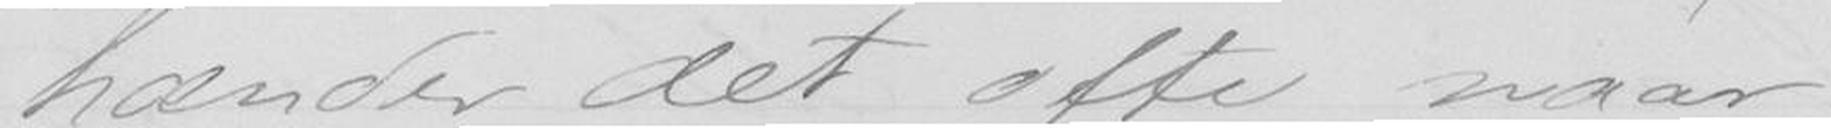hænder det ofte naar
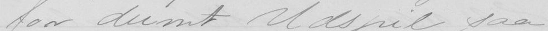for dumt Udspil, saa
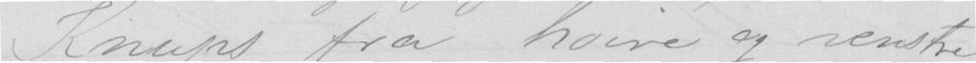Knups fra høire og venstre
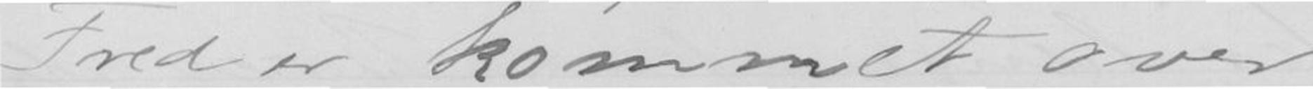Fred er kommet over
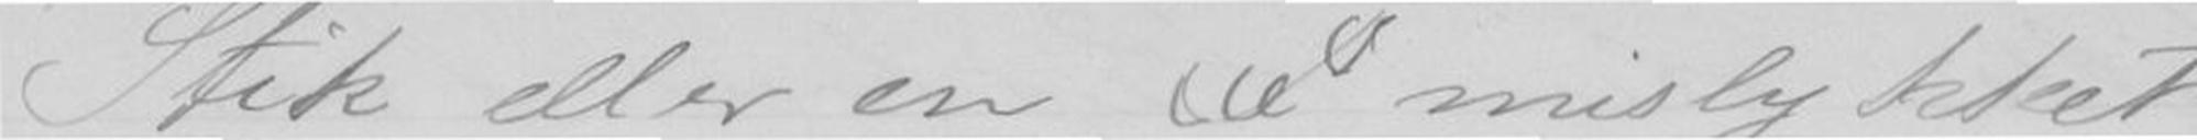Stik eller en mislykket
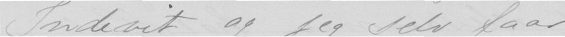Indevit og jeg selv faar
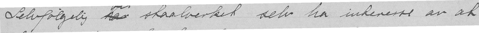Selvfølgelig har staalverket selv ha interesse av at
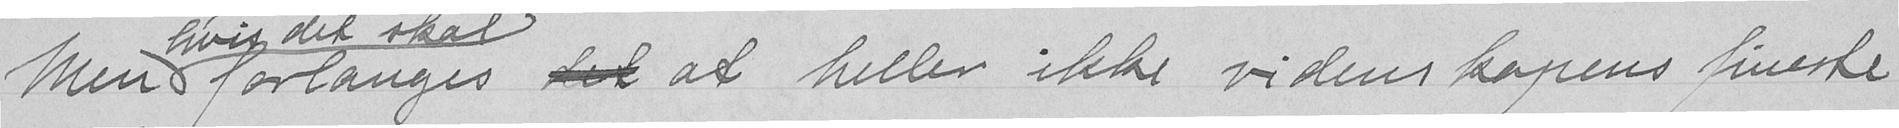Men forlanges det at heller ikke videnskapens fineste
